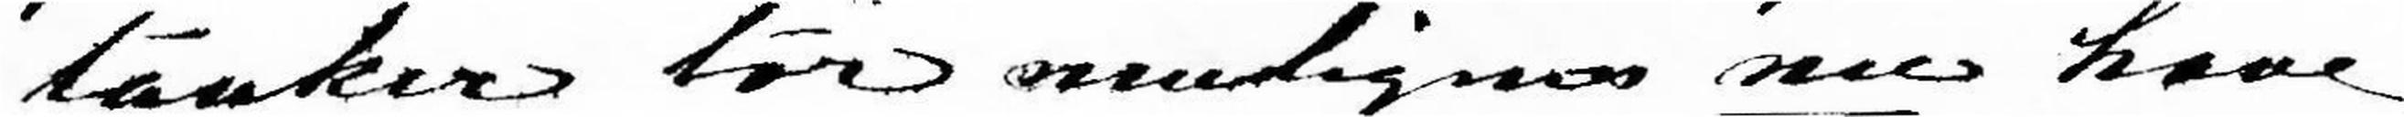tanker tør muligens nu have
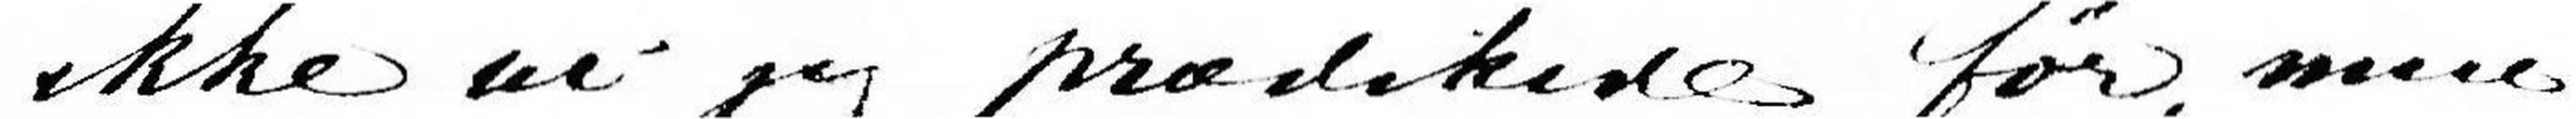ikke at jeg predikede før,
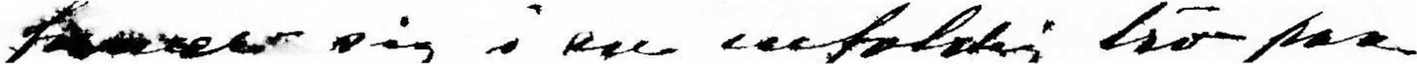formere sig i en enfoldig tro paa
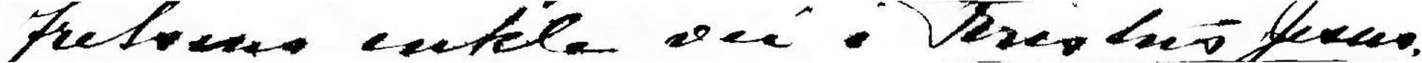frelsens enkle vei i Kristus Jesus.
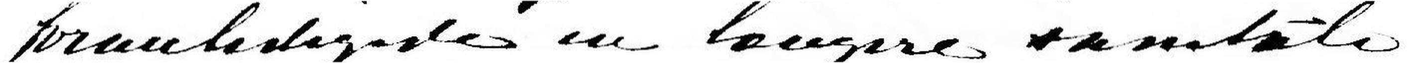foranledigede en længere samtale
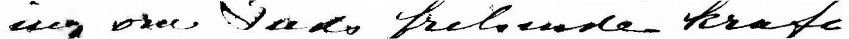ing om Guds frelsende kraft
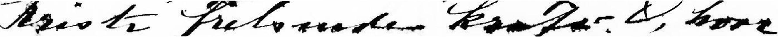Kristi frelsende kraft. O, hvor
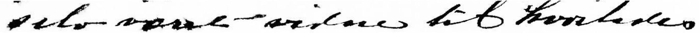selv været vidne til hvorledes
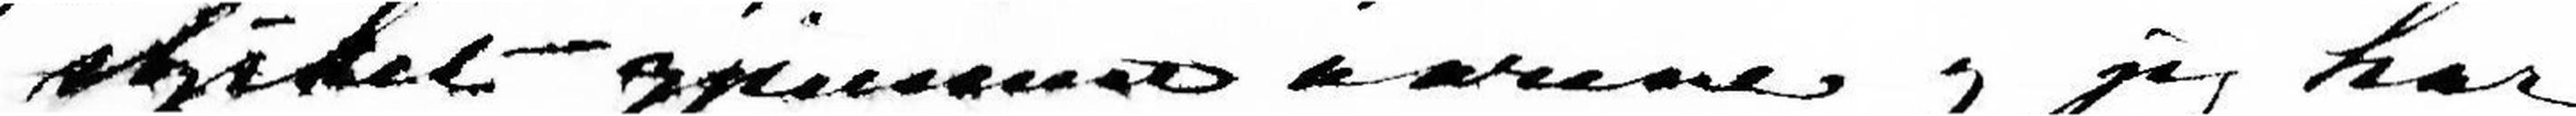styrket gjennem aarene og jeg har
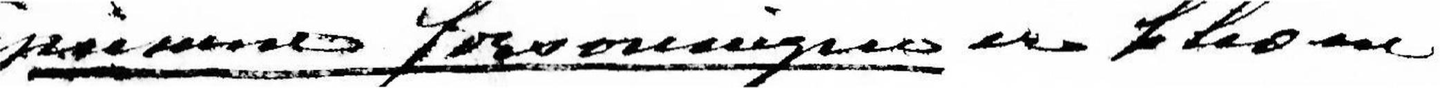Gjennem forsoningen er blevne
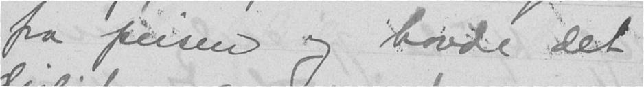fra peisen og havde det
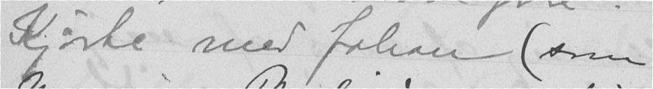Kjørte med Johan (som
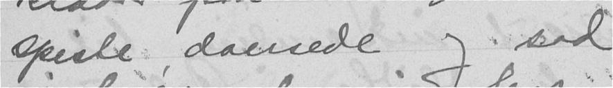spiste dernede og sad
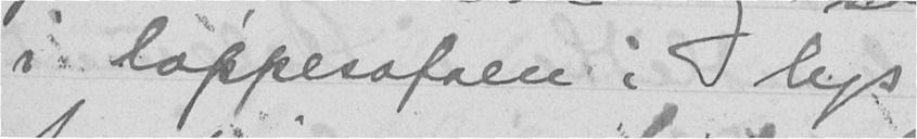i lappesofaen i lys
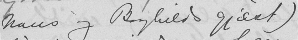hans og Borghilds gjæst)
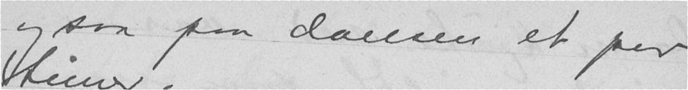og saa paa dansen et par
